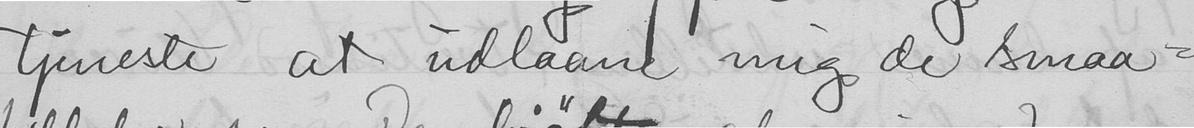tjeneste at udlaane mig de smaa-
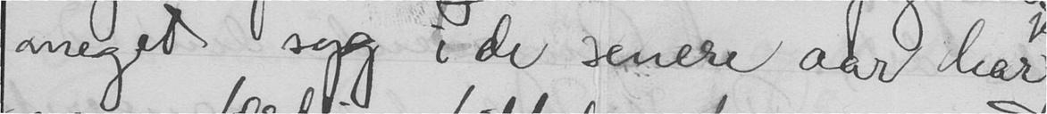meget syg e de senere aar har
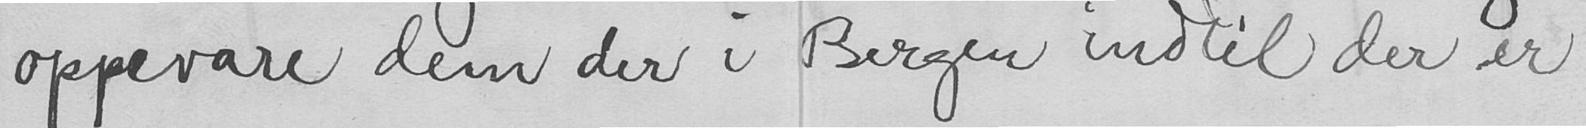oppevare dem der i Bergen indtil der er
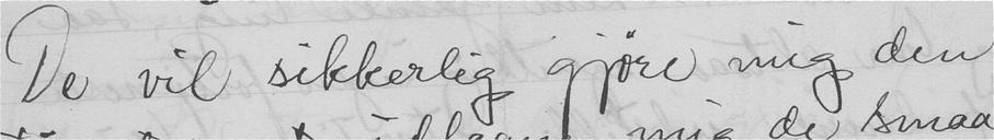De vil sikkerlig gjøre mig den
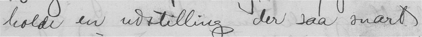holde en udstilling der saa snart
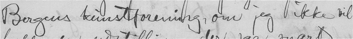Bergens kunstforening, om jeg ikke vil
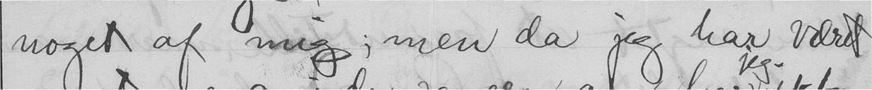noget af mig; men da jeg har været
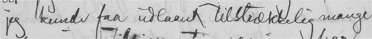jeg kunde faa udlaant "tilstrækkelig mange
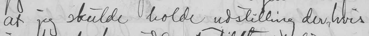at jeg skulde holde udstilling der, hvis
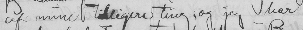af mine tidligere ting; og jeg har
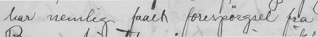har nemlig faaet forespørgsel fra
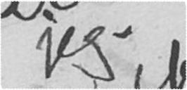jeg
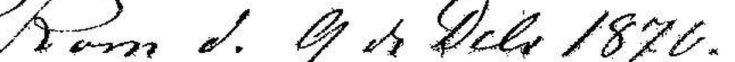Rom d. 9 de Dcbr 1870.
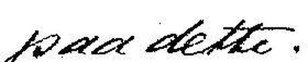paa dette.
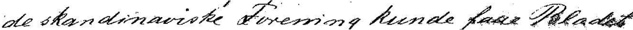de skandinaviske Forening kunde faae Bladet
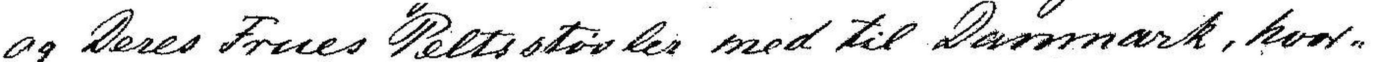og Deres Frues Peltsstøvler med til Danmark, hvor-
